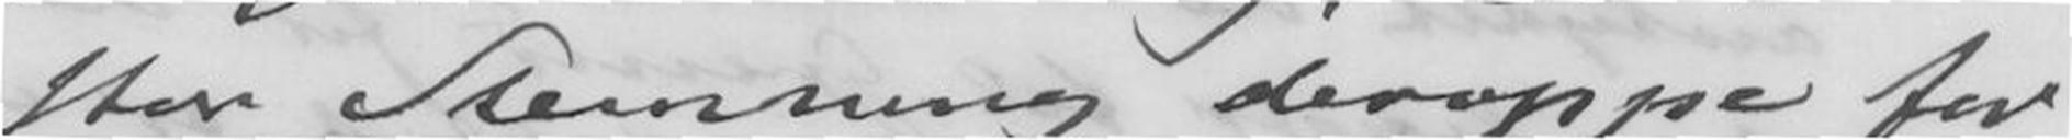stor Stemning deroppe for
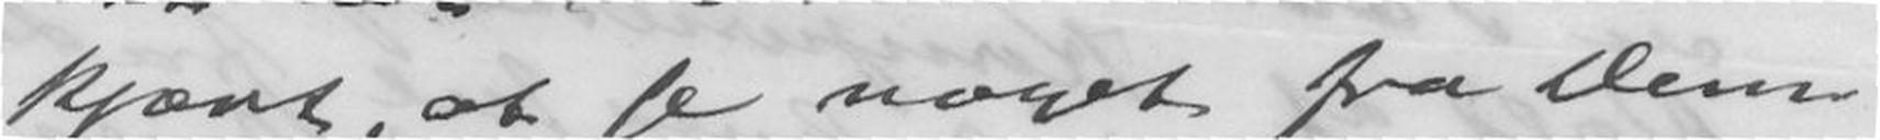kjært, at se noget fra Dem
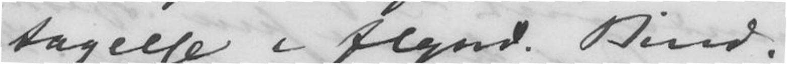tagelse i flgnd. Bind.
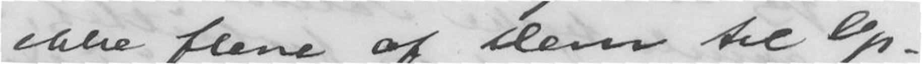ikke flere af Dem til Op-
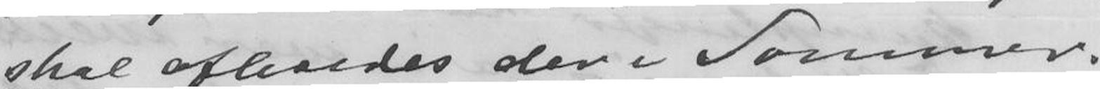skal afholdes der i Sommer.
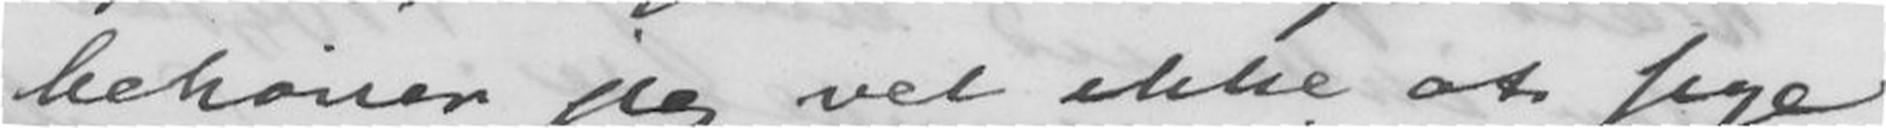behøver jeg vel ikke at sige
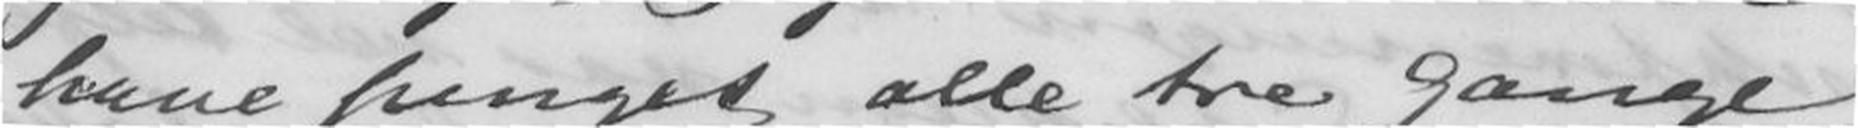have sunget alle tre Gange.
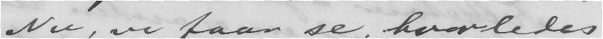Nu, vi faar se, hvorledes
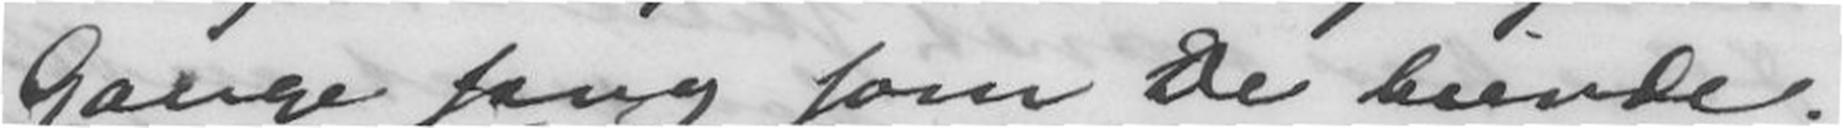Gange sang som De burde
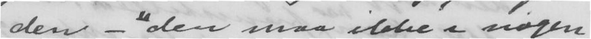den - "den maa ikke i nogen
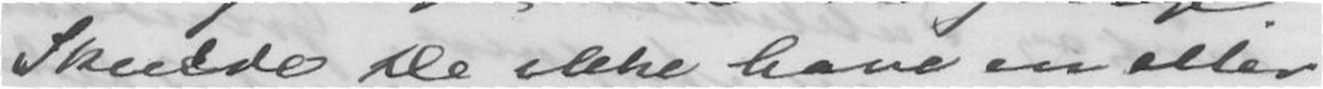Skulde De ikke have en eller
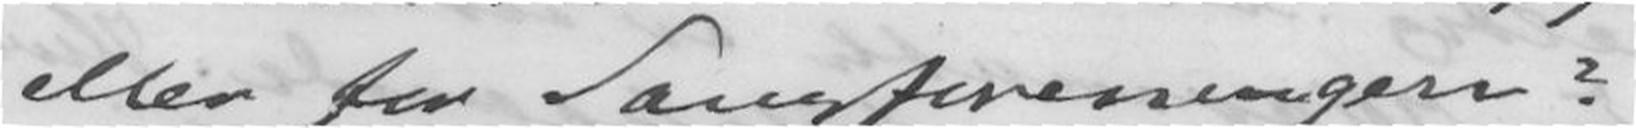Mer for Sangforeningen?
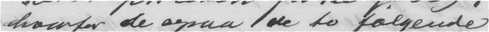hvorfor de ogsaa de to følgende
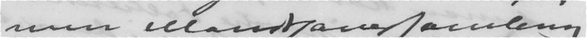min Mandsangsamlingen
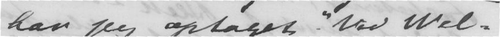har jeg optaget "Ved Wel-
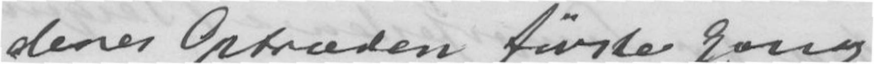deres Optræden første Gang,
